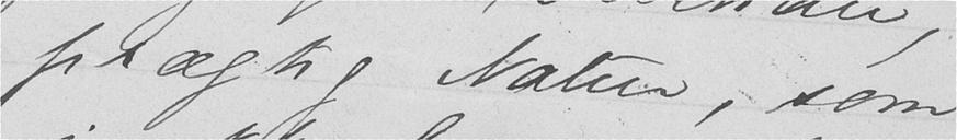prægtig Natur, som
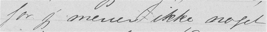for jeg mener ikke noget
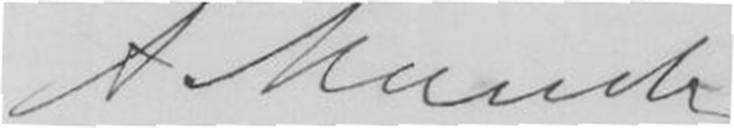A. Munch.
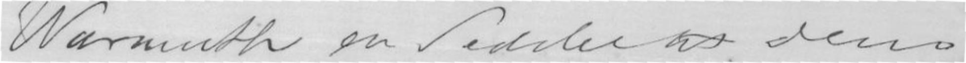Warmuth en Seddel til dens
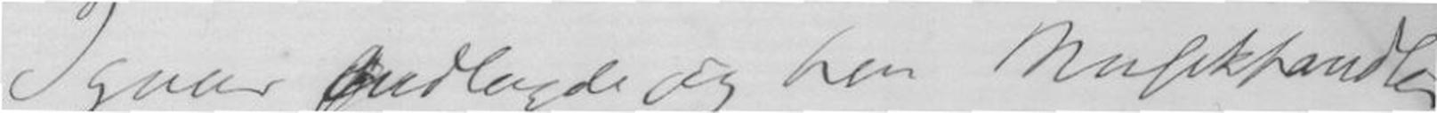I gaar andlagde jeg herr Musikhandler
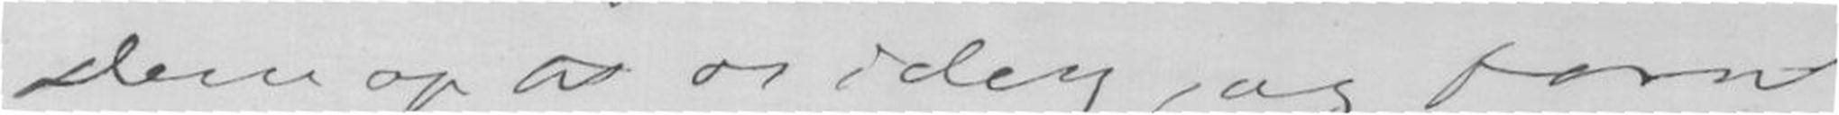Dem op til os idag, og forad
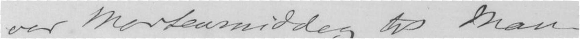vor Mortenmiddag til Man-
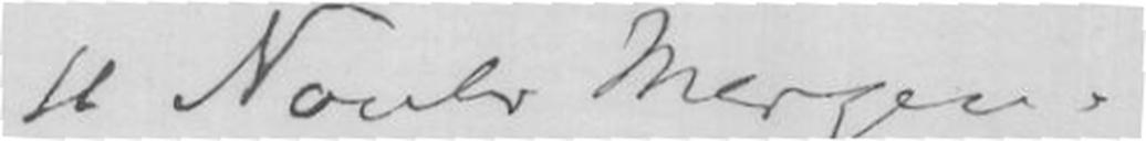4 Novbr. Bergen.
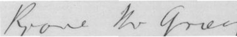Kjære Hr. Grieg
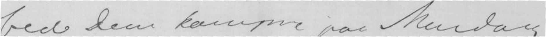bed Dem komme paa Mandag
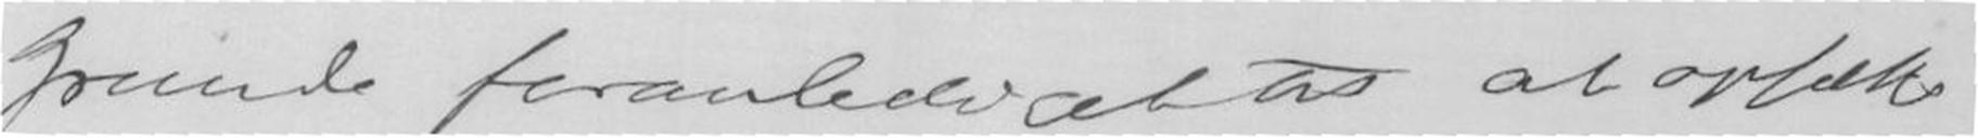Grunde foranlediget til at opsætte
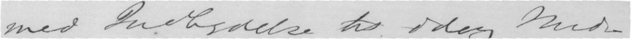med Indbydelse til idag Mid-
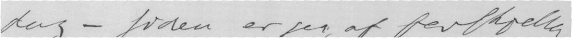dag - siden er jeg, af forskjellige
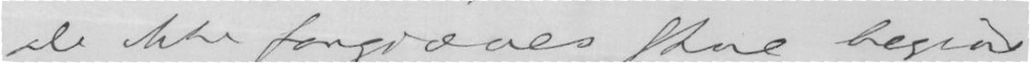De ikke forgjæves skal begive
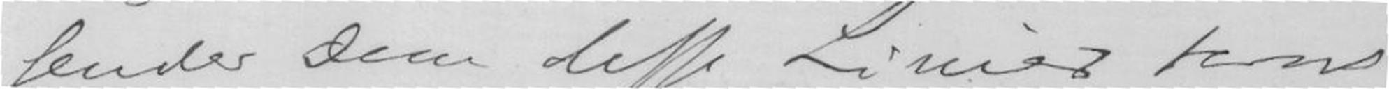sender Dem disse Linier forad
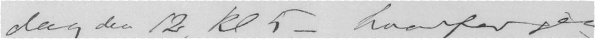dag den 12, kl 5 - hvorfor jeg
